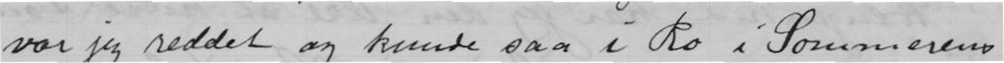var jeg reddet og kunde saa i Ro i Sommerens
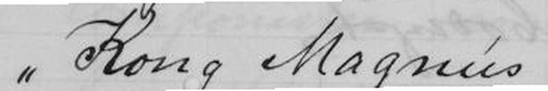"Kong Magnus!"
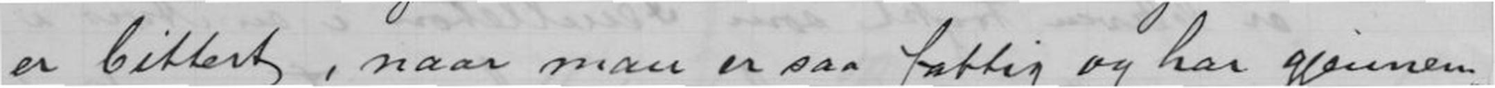er bittert, naar man er saa fattig og har gjennem-
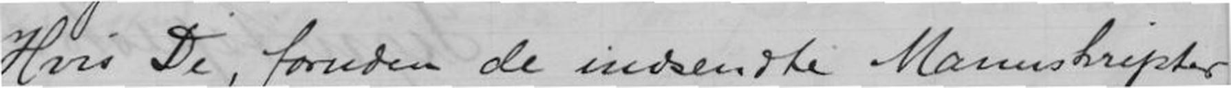Hvis De, foruden de indsendte Manuskripter
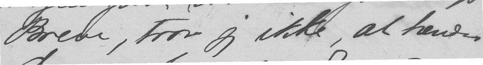Breve, tror jeg ikke at hendes
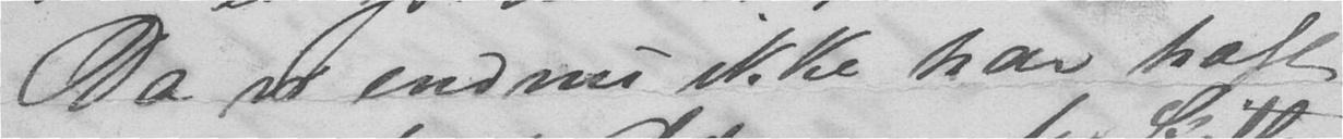Da vi endnu ikke har haft
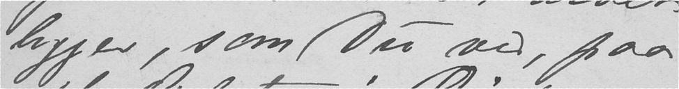ligger, som Du ved, paa
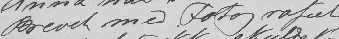Brevet med Fotografiet
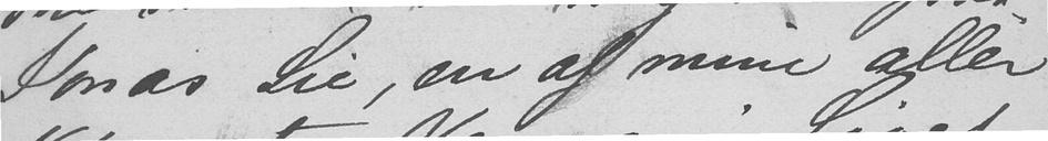Jonas Lie, en af mine aller
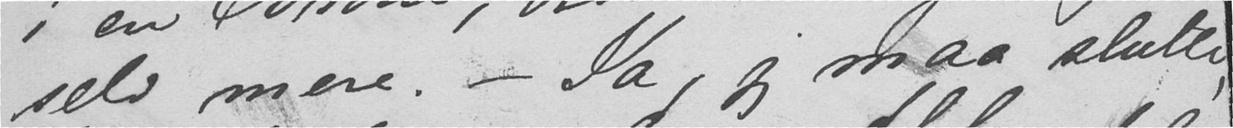selv mere. - Ja, jeg maa slutte,
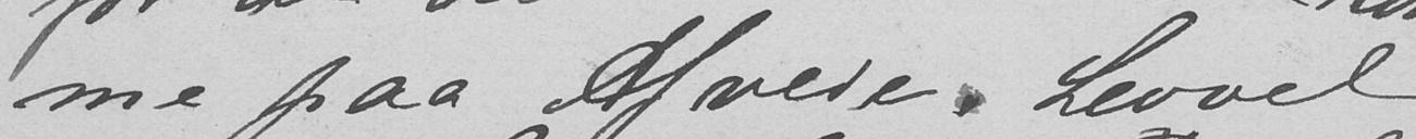me paa Afveie. Levvel
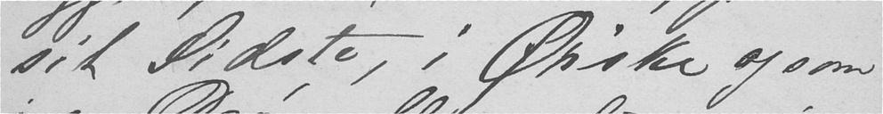sit Sidste, i Ørske og som
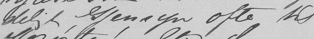eligt Gjensyn ofte til
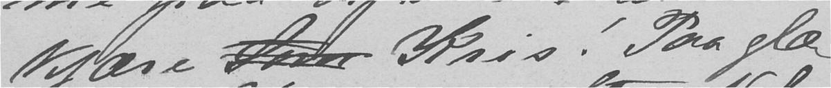Kjære Gate Kris. Paa glæ-
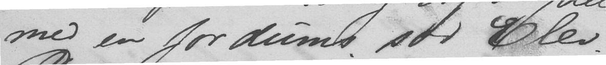med en fordums sød Elev.
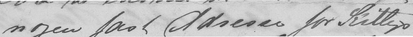nogen fast Adresse for Kittys
